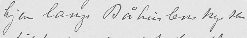hjem langs Båhuslenskysten
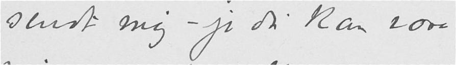sendt mig — jo du kan være
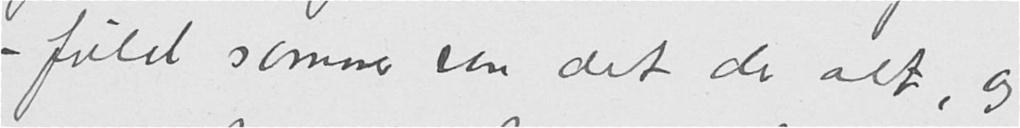– fuld sommer var det der alt, og
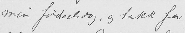min fødselsdag, og takk for
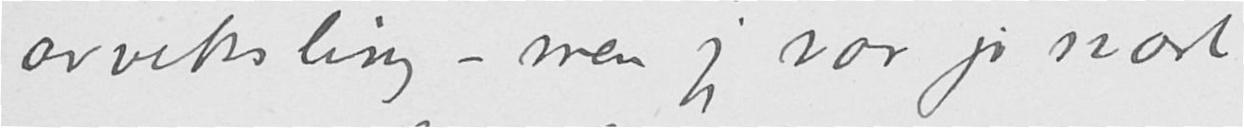avviksling - men jeg var jo svært
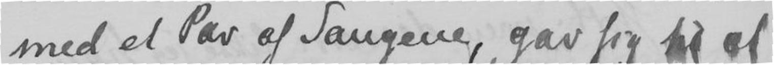med et Par af Sangene, gav sig til at
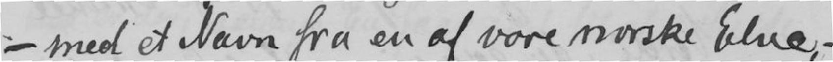-med et Navn fra en af vore norske Elve, -
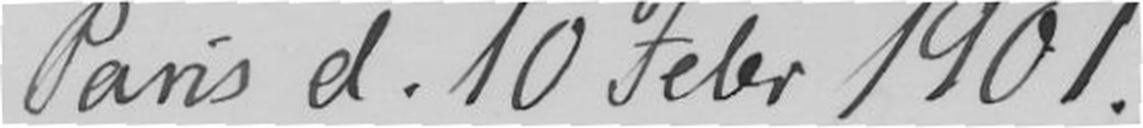Paris d. 10 Febr 1901.
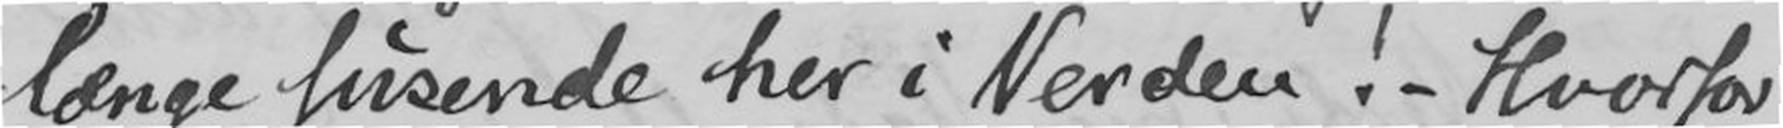længe susende her i Verden! - Hvorfor
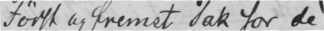Først og fremst Tak for de
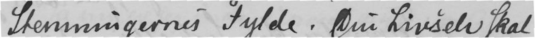Stemningernes Fylde. Din Livselv skal
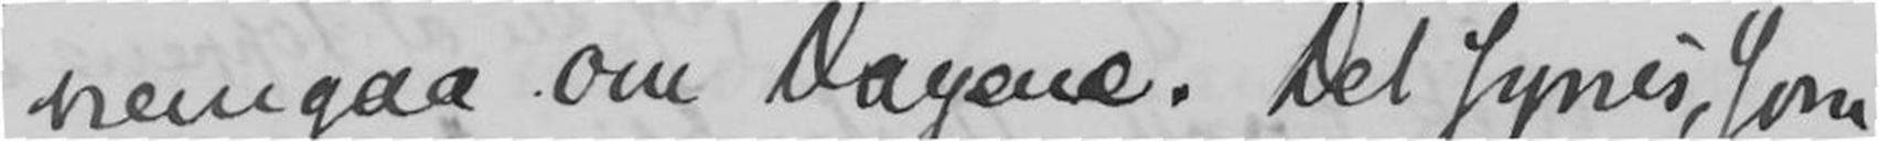nemgaa om Dagene. Det synes som
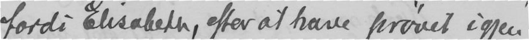fordi Elisabeth, efter at have prøvet igjen
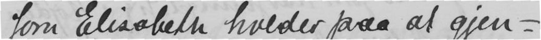som Elisabeth holder paa at gjen-
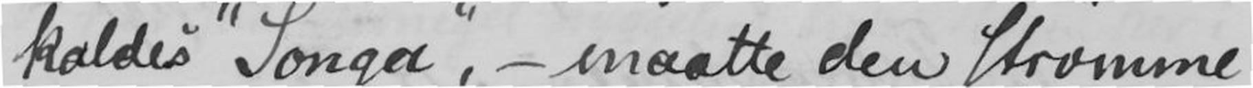kaldes "Songa", - maatte den strømme
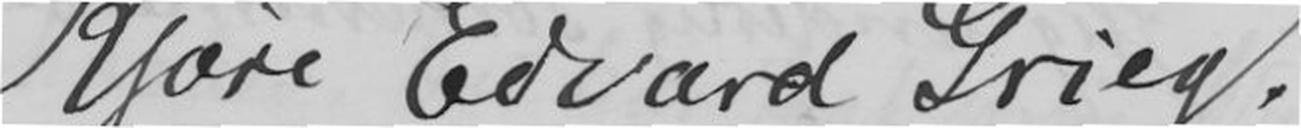Kjære Edvard Grieg.
